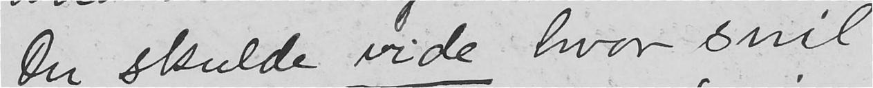Du skulde vide hvor snil
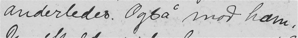anderledes. Også mod ham,
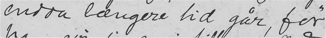endda længere tid går, før
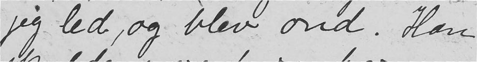jeg led, og blev ond. Han
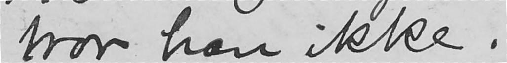tror han ikke.
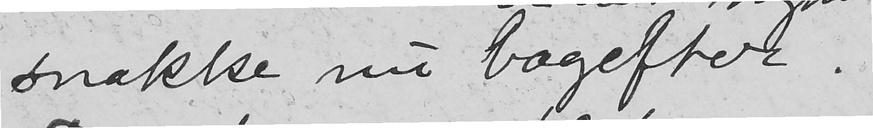snakke nu bagefter.
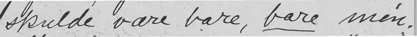skulde være bare, bare min.
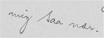mig saa nær.
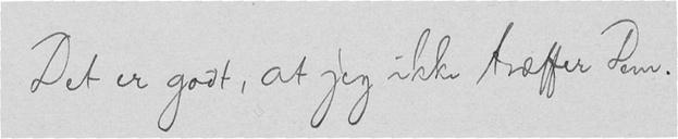Det er godt, at jeg ikke træffer Dem.
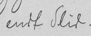endt Slid.
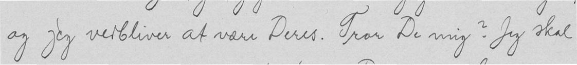og jeg vedbliver at være Deres. Tror De mig? Jeg skal
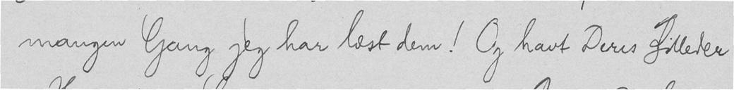mangen Gang jeg har læst dem! Og havt Deres Billeder
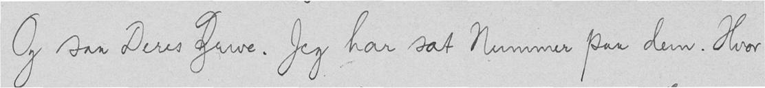Og saa Deres Breve. Jeg har sat Nummer paa dem. Hvor
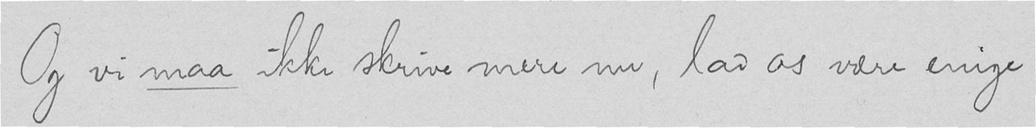Og vi maa ikke skrive mere nu, lad os være enige
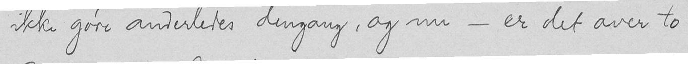ikke gøre anderledes dengang, og nu - er det over to
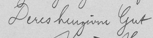Deres hengivne Gut
